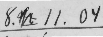8. 11.04
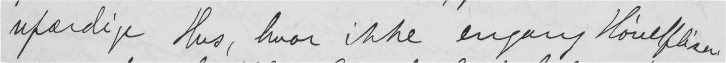ufærdige Hus, hvor ikke engang Høvelflisen
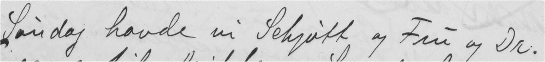Søndag havde vi Schjøtt og Fru og Dr.
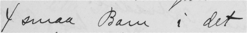4 smaa Barn i det uoppe saasast
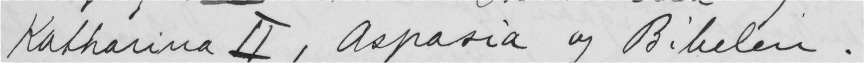Katharina II, Aspasia og Bibelen.
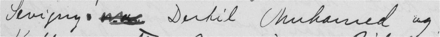Sevigny.  Dertil Muhamed og
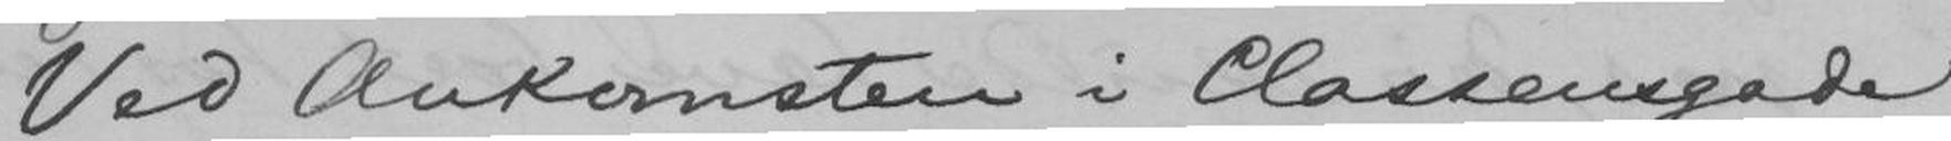Ved Ankomsten i Classensgade
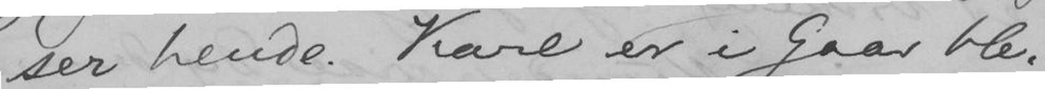ser hende. Karl er i Gaar ble-
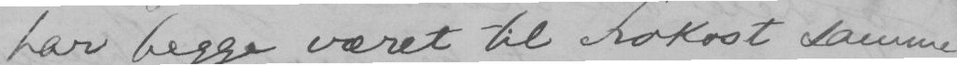har begge været til Frokost samme
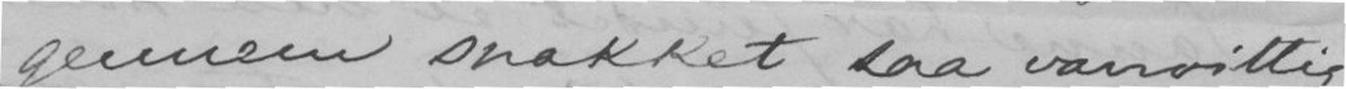gennem snakket saa vanvittig
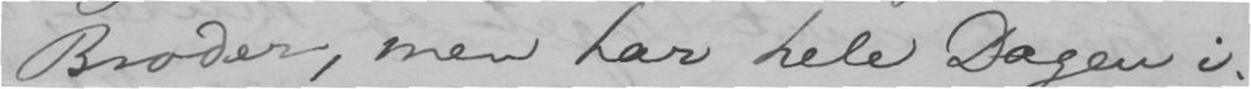Broder, men har hele Dagen i-
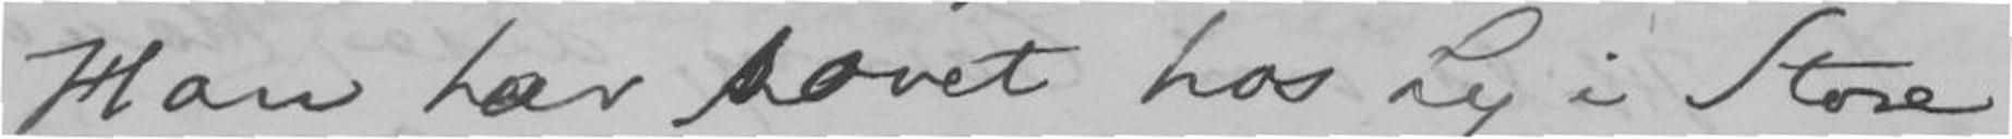Han har sovet hos Ly i Store
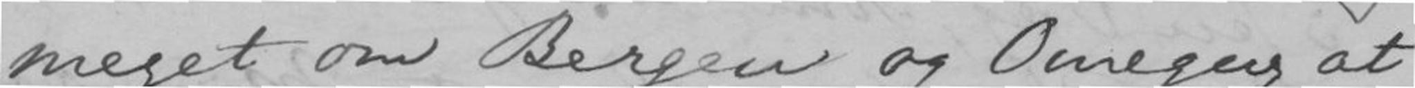meget om Bergen og Omegne at
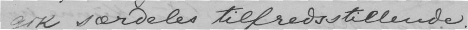gik særdeles tilfredsstillende.
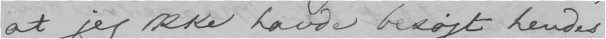at jeg ikke havde besøgt hendes
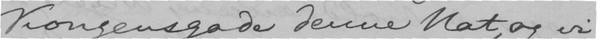Kongensgade denne Nat, og vi
